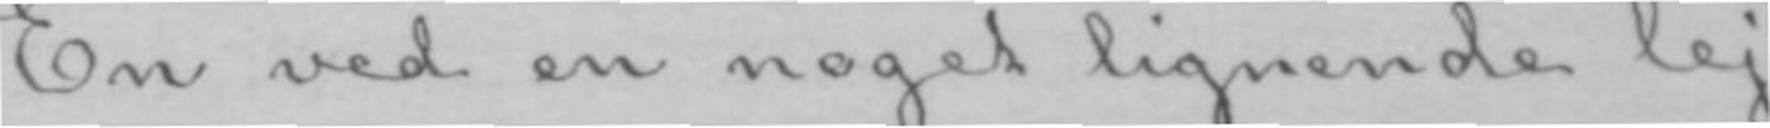En ved en noget lignende lej-
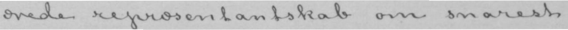ærede repræsentantskab om snarest
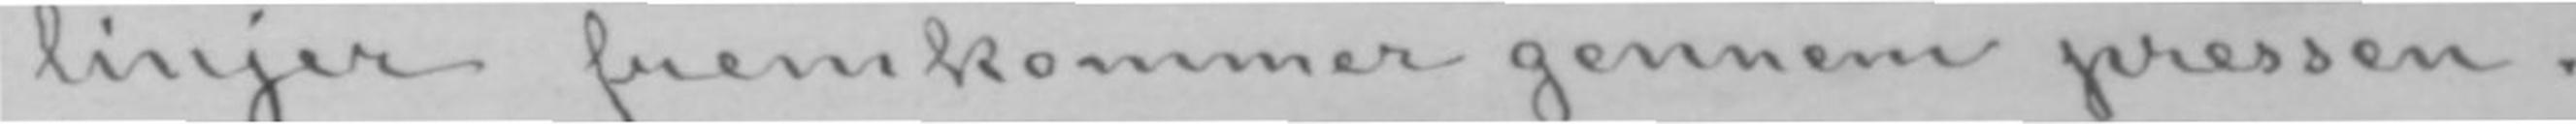linjer fremkommer gennem pressen.
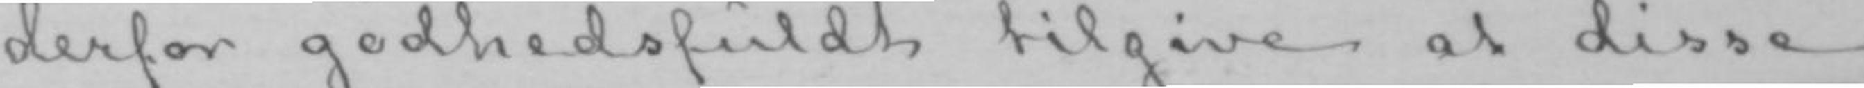derfor godhedsfuldt tilgive at disse
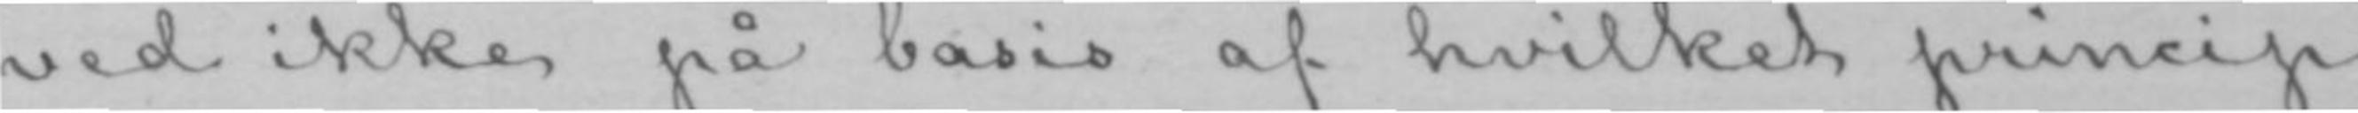ved ikke på basis af hvilket princip
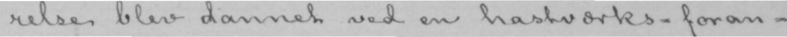relse blev dannet ved en hastværks-foran-
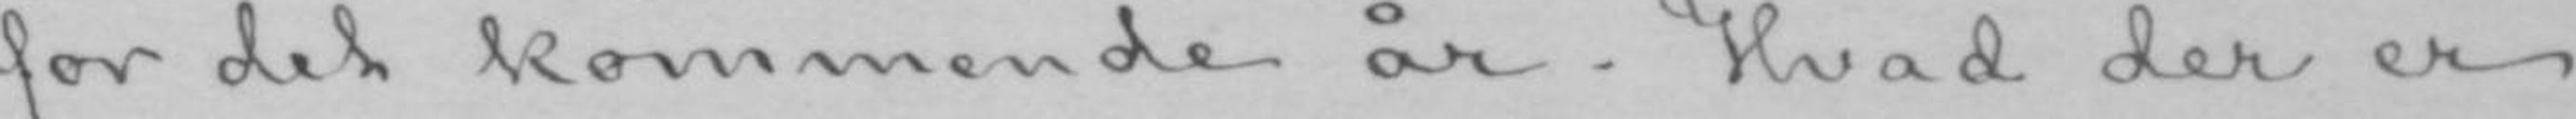for det kommende år. Hvad der er
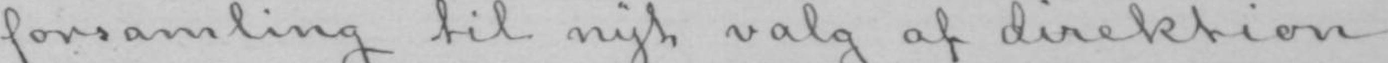forsamling til nyt valg af direktion
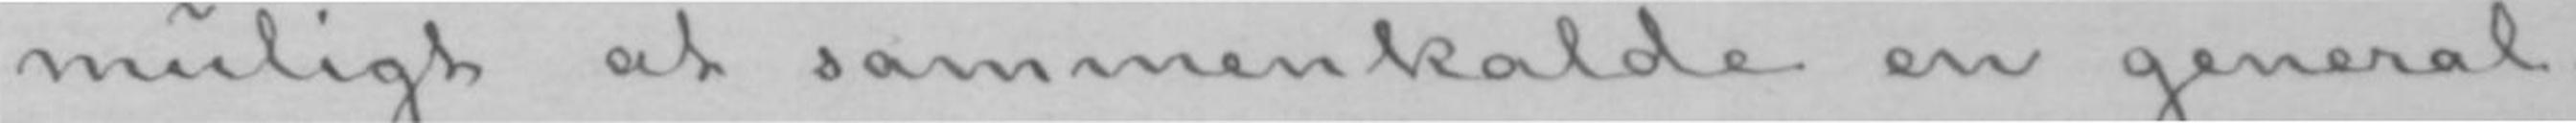muligt at sammenkalde en general-
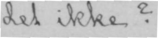det ikke?
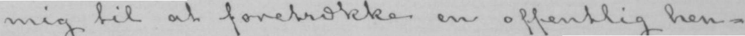mig til at foretrække en offentlig hen-
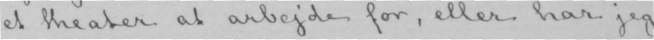et theater at arbejde for, eller har jeg
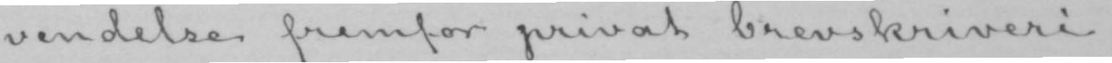vendelse fremfor privat brevskriveri.
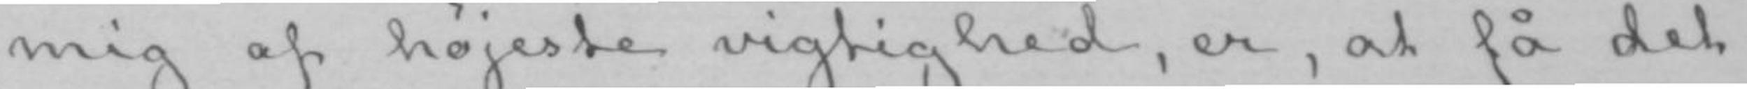mig af højeste vigtighed, er, at få det
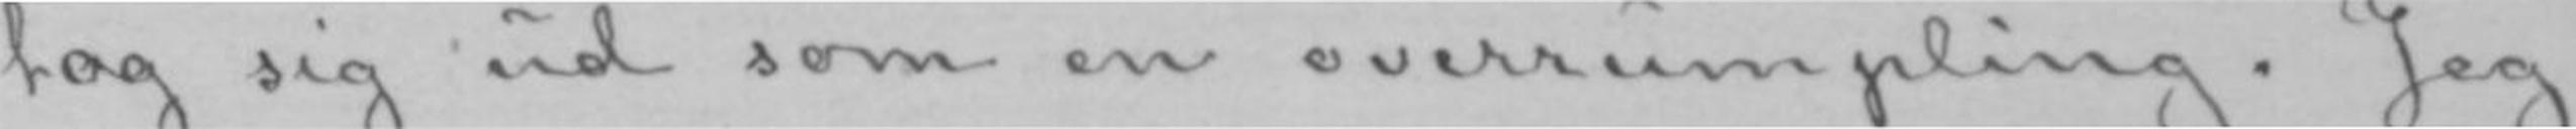tog sig ud som en overrumpling. Jeg
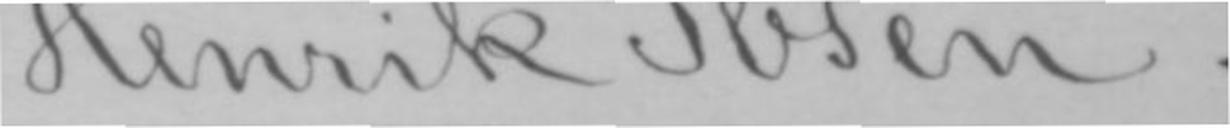Henrik Ibsen.
